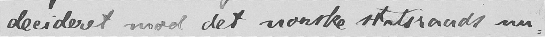decideret mod det norske statsraads nu-
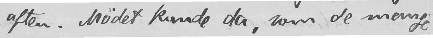aften. Mødet kunde da, som de mange
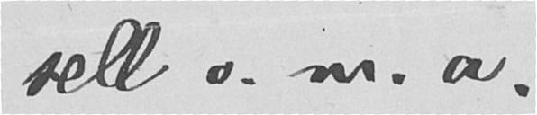sell o. m. a.
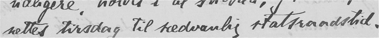sættes tirsdag til sædvanlig statsraadstid.
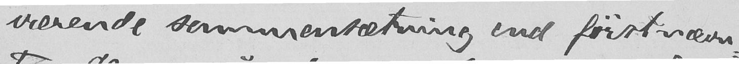værende sammensætning end førstnævn-
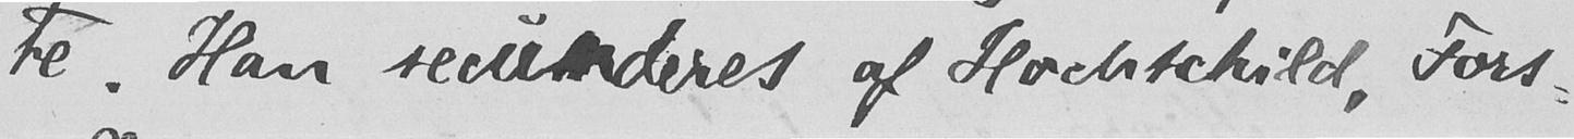te. Han secunderes af Hochschild, Fors-
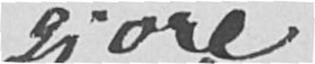gjøre
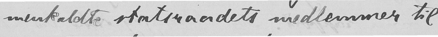menkaldte statsraadets medlemmer til
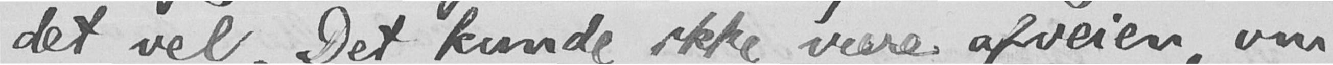det vel. Det kunde ikke være afveien, om
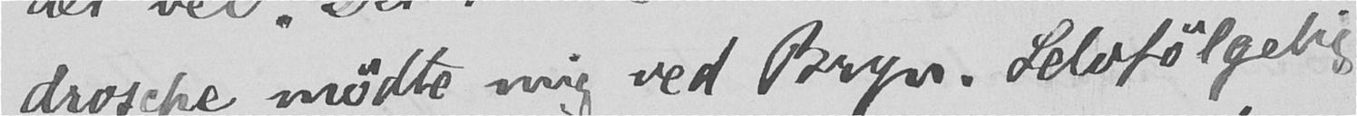drosche mødte mig ved Bryn. Selvfølgelig
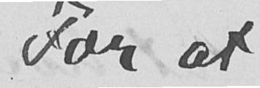For at
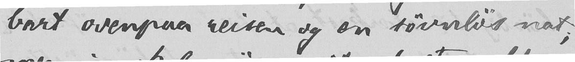bart ovenpaa reisen og en søvnløs nat;
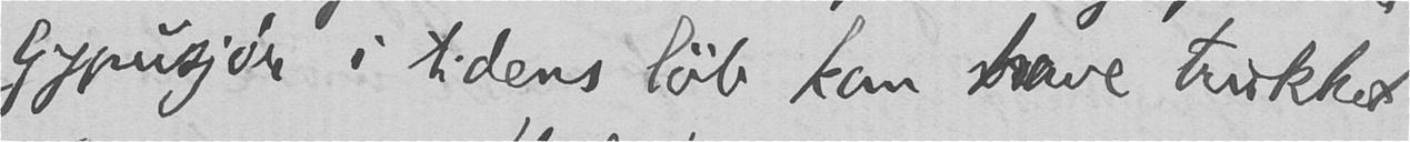Gypusjór i tidens løb kan have trukket
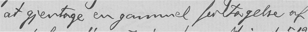at gjentage en gammel feiltagelse af
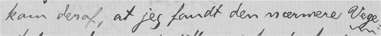kom deraf, at jeg fandt den nærmere Vege-
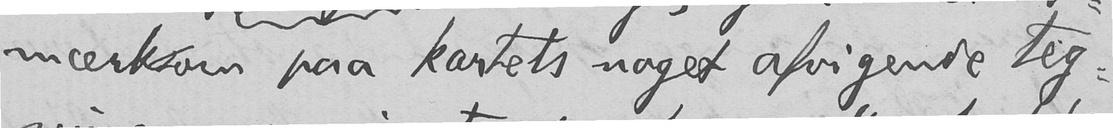mærksom paa kartets noget afvigende teg-
